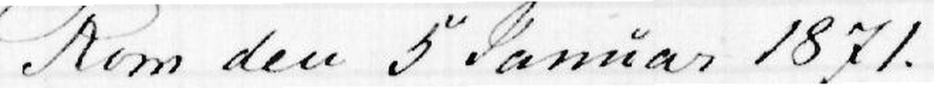Rom den 5 Januar 1871.
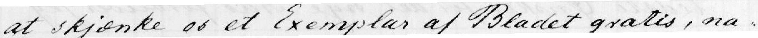at skjænke os et Exmplar af Bladet gratis, na-
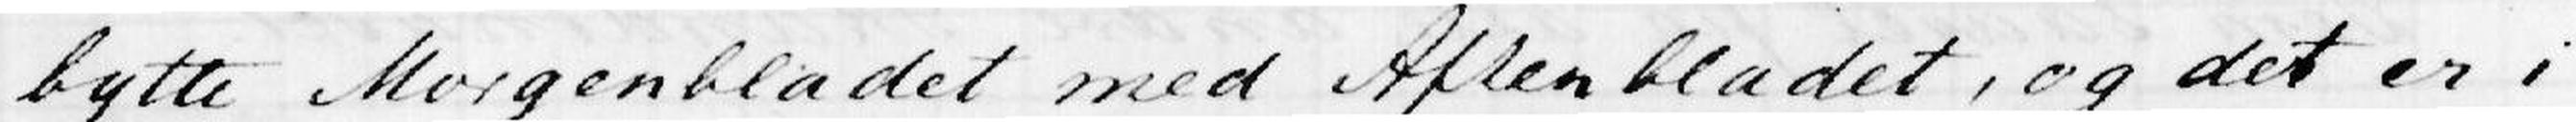bytte Morgenbladet med Aftenbladet, og det er i
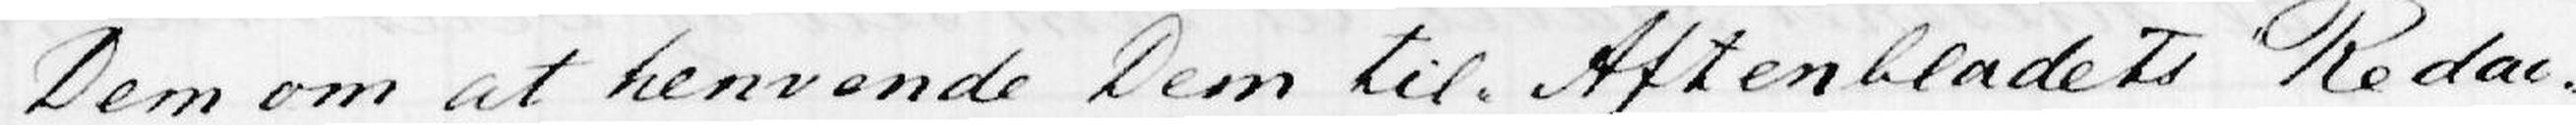Dem om at henvende Dem til Aftenbladets Redac-
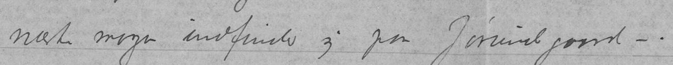næste morgen indfinder sig paa Jørundgaard –.
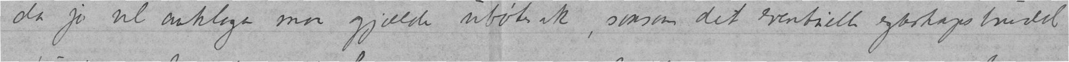da jo vel anklagen maa gjælde ubotesak, saasom det eventuelle egteskapsbrudd
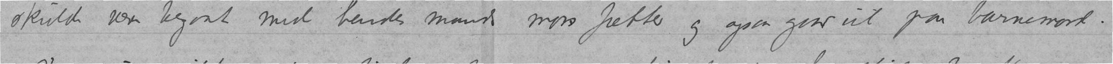skulde være begaat med hendes mands mors fætter og ogsaa gaar ut paa barnemord.
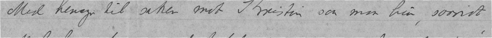Med hensyn til saken mot Kristin saa maa hun saavidt
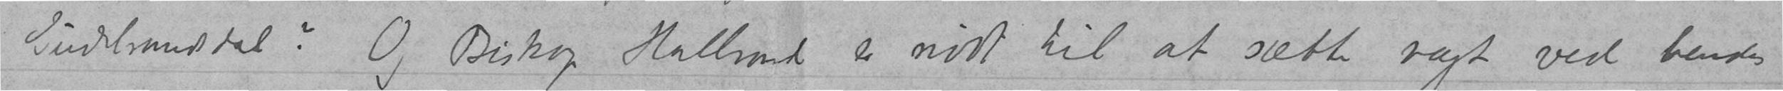Gudsbrandsdal? Og Biskop Hallvard er nødt til at sætte vagt ved hendes
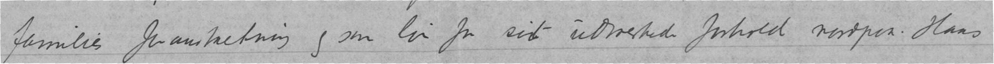families foranstaltning og som løn for sit udmerkede forhold nordpaa. Hans
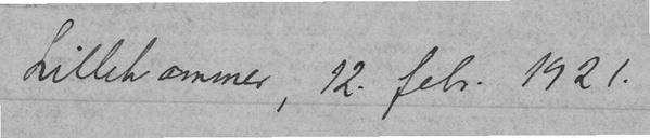Lillehammer, 12 febr. 1921.
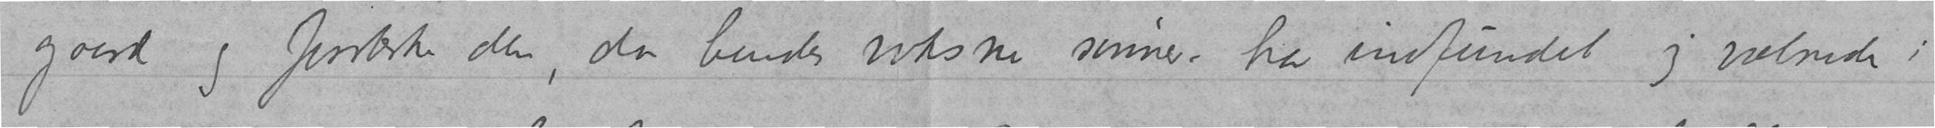gaard og forsterke den, da hendes voksne sønner har indfundet sig vælnede i
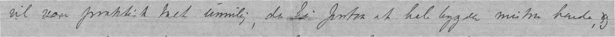vil være praktisk talt umulig, da hun forstaar at hele bygden mistror hende, og
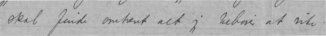skal finde omtrent alt jeg behøver at vite.
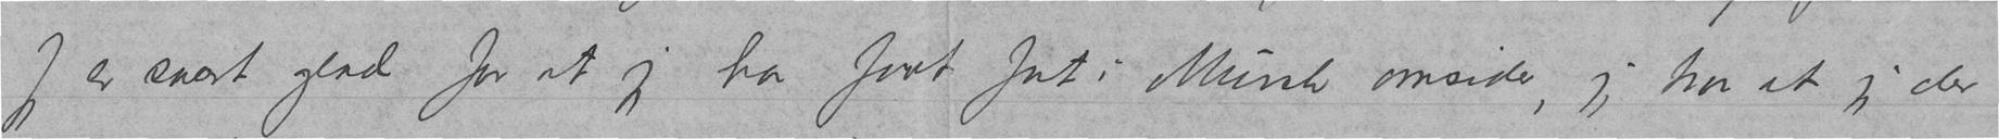Jeg er svært glad for at jeg har faat fat i Munch omsider, jeg tror at jeg der
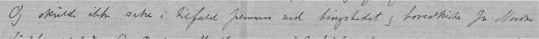Og skulde ikke saken i tilfælde fremmes ved tingstedet og hovedkirken for Nordre
